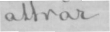attrår.
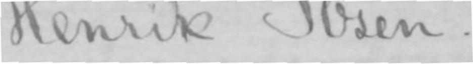Henrik Ibsen.
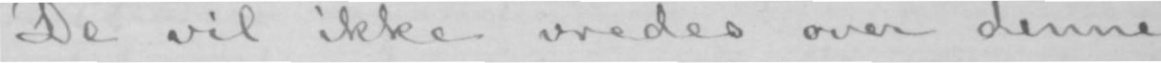De vil ikke vredes over denne
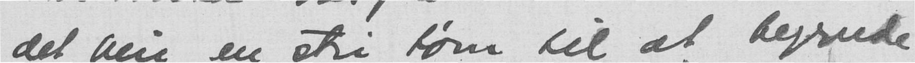det blir en stri tørn til at begynde
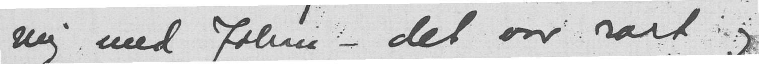mig med Johan - det var rart og
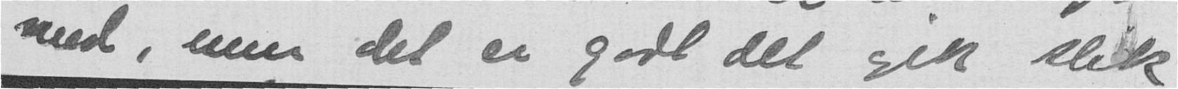med, men det er godt det gik slik
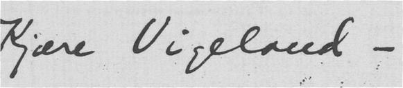Kjære Vigeland-
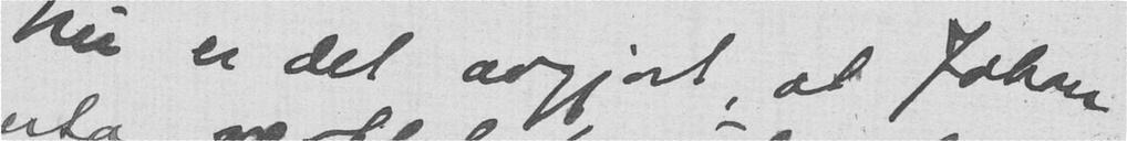her er det avgjort, at Johan
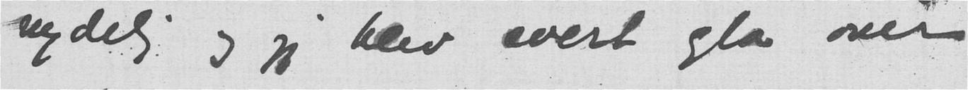nydelig og jeg blev svert gla over
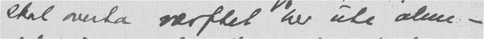skal overta verftet her ute alene-
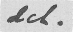det.
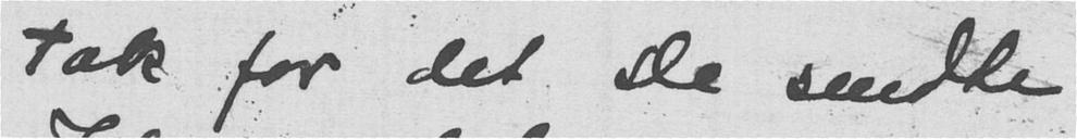tak for det De sendte
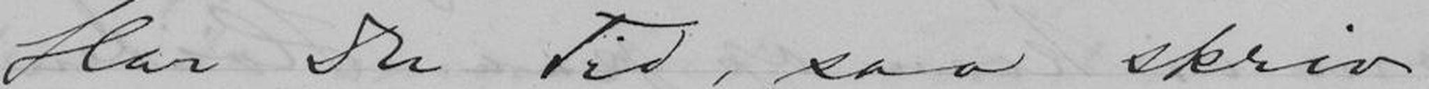Har Du Tid, saa skriv
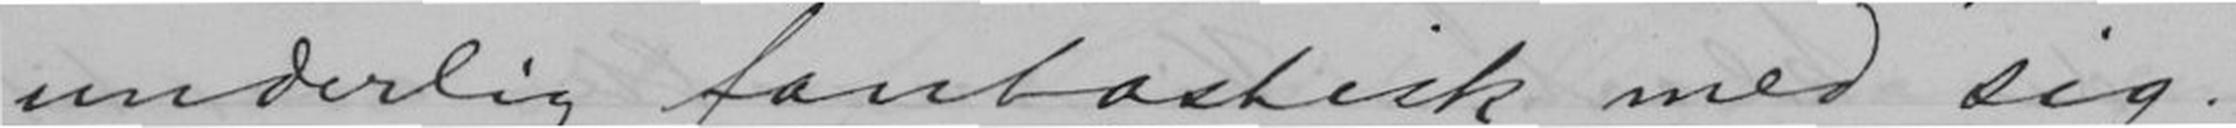underlig fantastisk med sig.
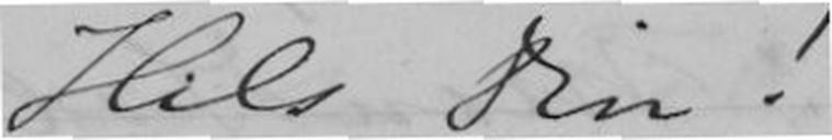Hils Din!
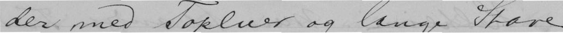der med Topluer og lange Stave
